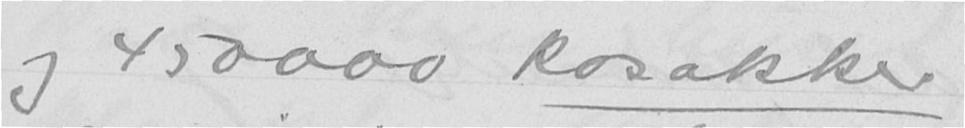og 450000 kosakker
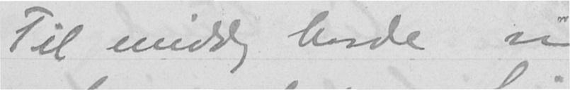Til middag havde vi
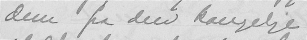dem fra den kongelige
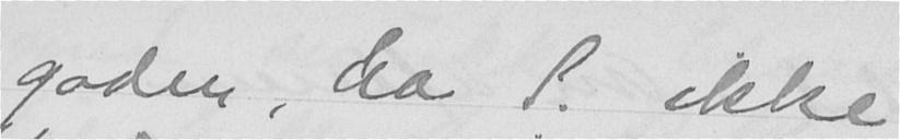gaden, da S. ikke
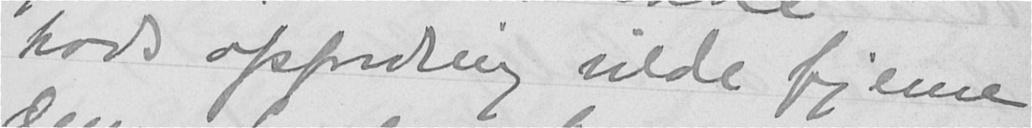trods opfordring vilde fjerne
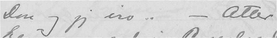Don og jeg iro. - Atter
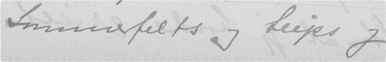Sommerfelts og Seips og
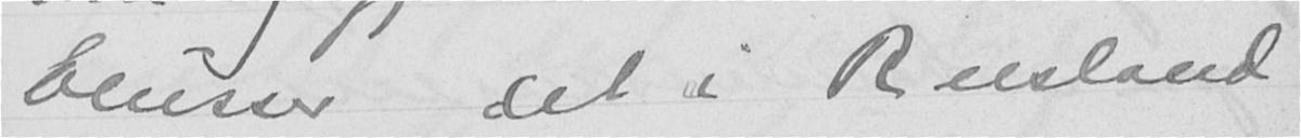blusser det i Rusland
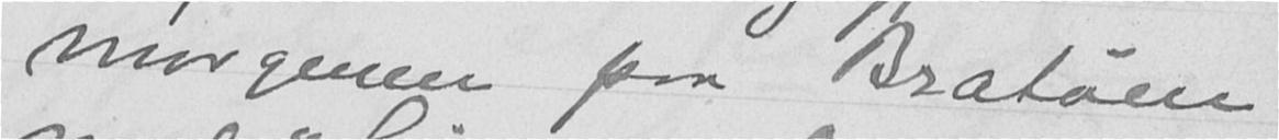morgenen paa Bratøen
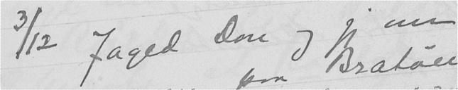3/12 Jaged Don og jeg om
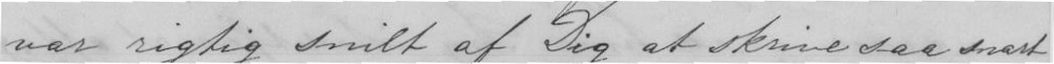var rigtig snilt af Dig at skrive saa snart
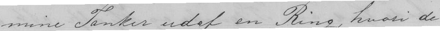mine Tanker udaf en Ring, hvori de
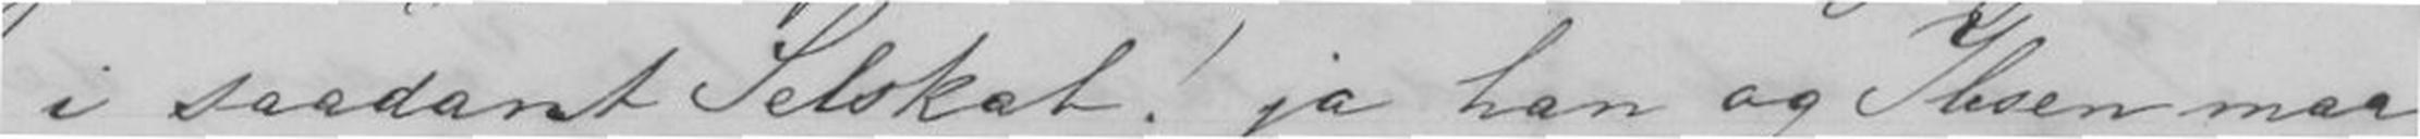i saadant Selskab! ja han og Ibsen maa
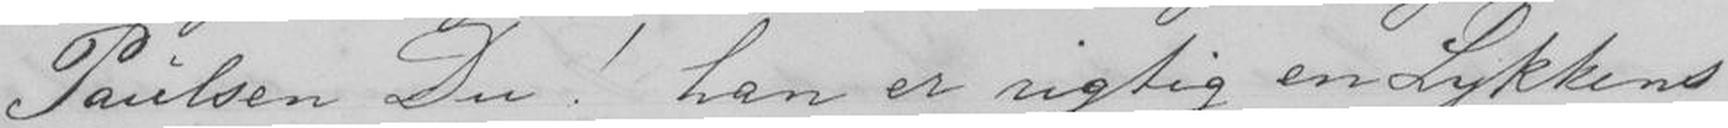Paulsen Du! han er rigtig en Lykkens
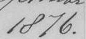1876
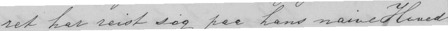ret har reist sig paa hans naive Hoved
